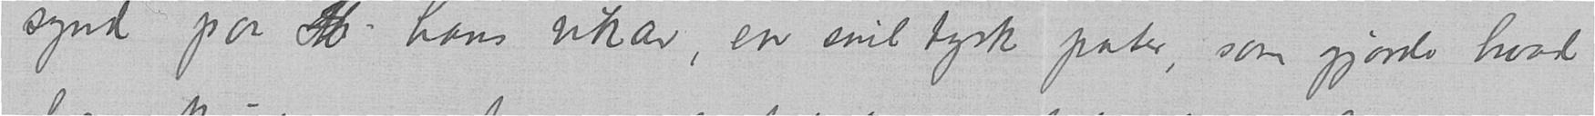synd paa  hans vikar, en snil tysk pater, som gjorde hvad
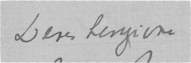Deres hengivne
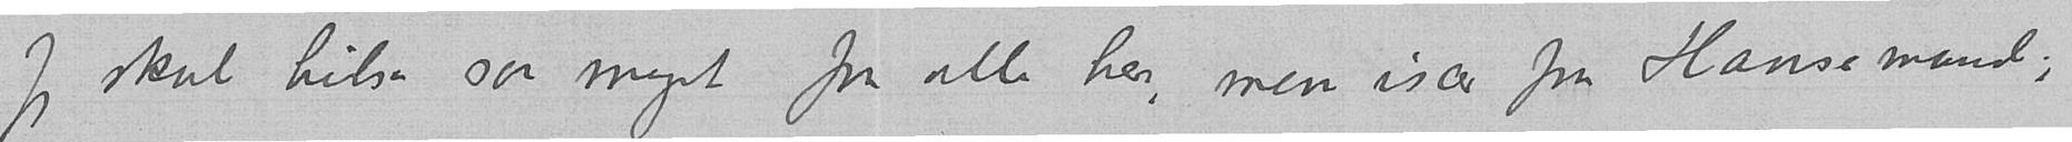Jeg skal hilse saa meget fra alle her, men især fra Hansemand;
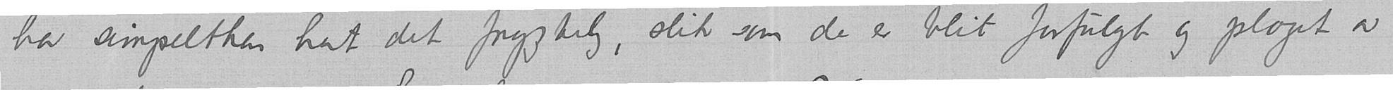har simpelthen hat det frygtelig, slik som de er blit forfulgt og plaget av
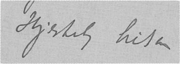Hjertelig hilsen
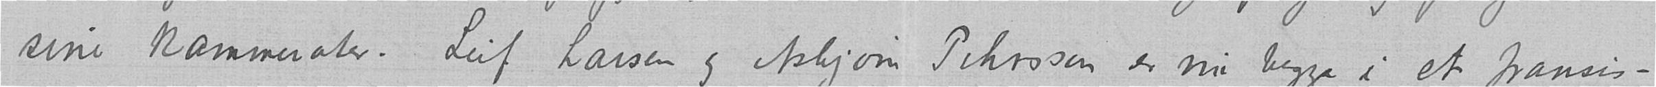sine kammerater. Leif Larsen og Asbjørn Pehrsson er nu begge i et fransis-
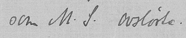som M.S. avslørte.
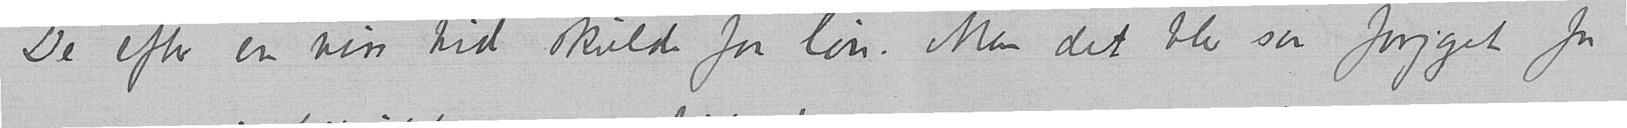De efter en viss tid skulde faa løn. Men det blev saa forjaget for
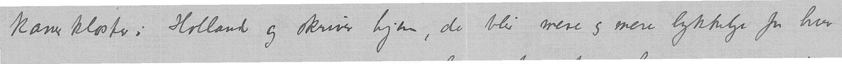kanerkloster i Holland og skriver hjem, de blir mere og mere lykkelige for hver
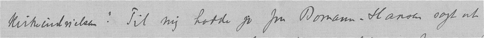kirkeindvielsen? Til mig hadde jo fru Bomann-Hansen sagt at
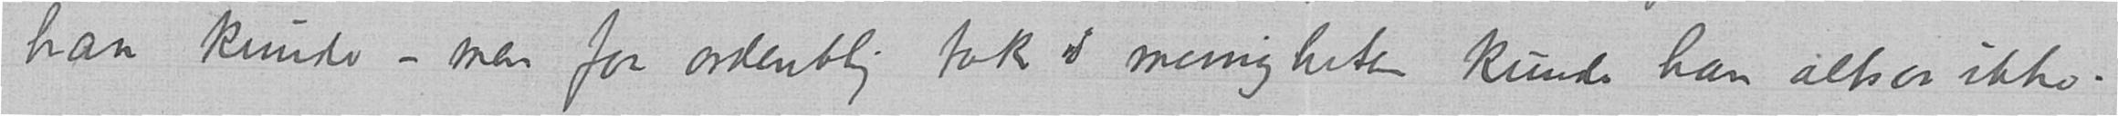han kunde – men faa ordentlig tak i menigheten kunde han altsaa ikke.
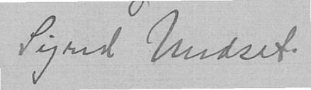Sigrid Undset.
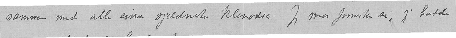sammen med alle sine sjeldneste klenodier. Jeg maa forresten si, jeg hadde
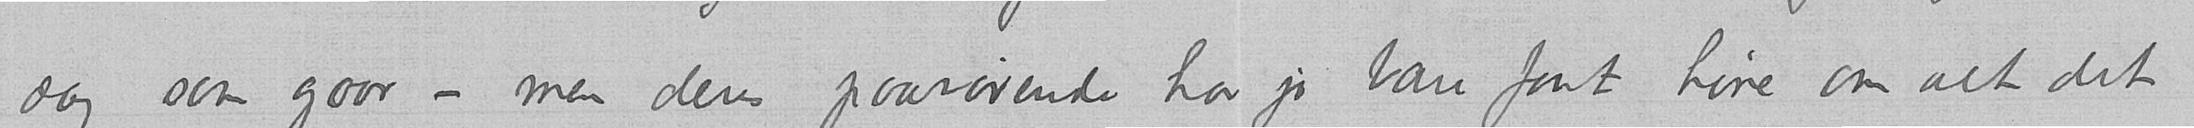dag som gaar – men deres paarørende har jo bare faat høre om alt det
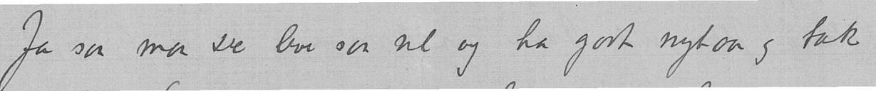Ja saa maa De leve saa vel og ha godt nytaar og tak
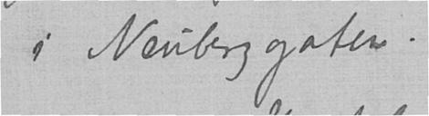i Neuberggaten.
